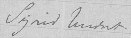Sigrid Undset.
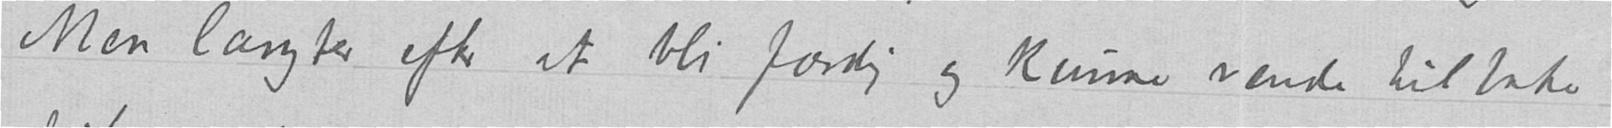Men længter efter at bli færdig og kunne vende tilbake
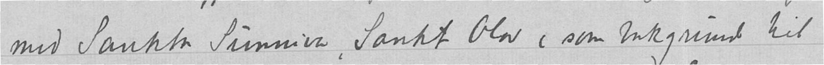med Sankta Sunniva, Sankt Olav (som bakgrund til
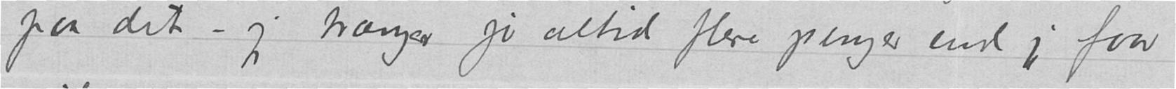paa det – jeg trænger jo altid flere penger end jeg faar
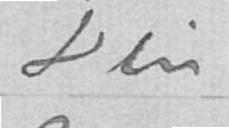Din
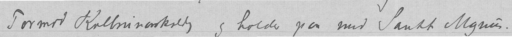Tormod Kolbrunarskald og holder paa med Sankt Magnus.
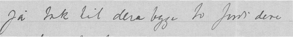Ja tak til dere begge to fordi dere
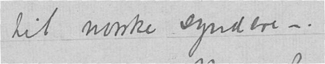til norske syndere –.
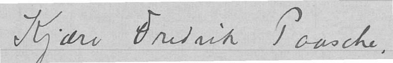Kjære Fredrik Paasche,
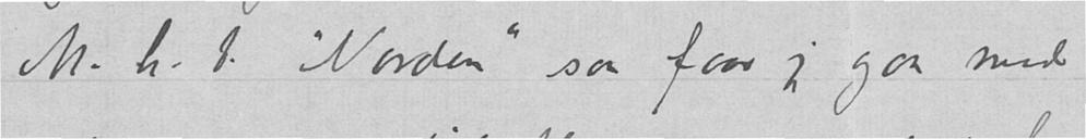M.h.t. "Norden" saa faar jeg gaa med
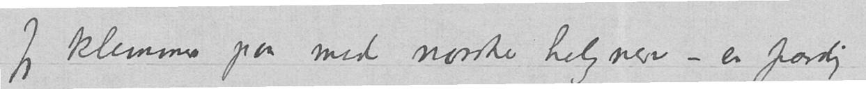Jeg klemmer paa med norske helgner - er færdig
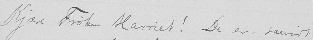Kjære Frøken Harriet! De er - saavidt
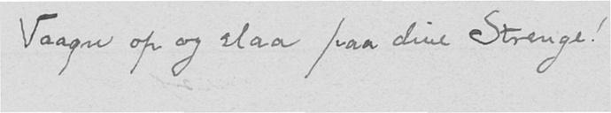Vaagn op og slaa paa dine Strenge!
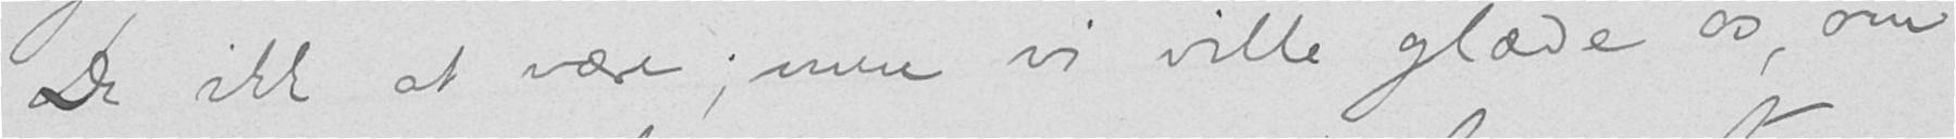De ikke at være; men vi ville glæde os, om
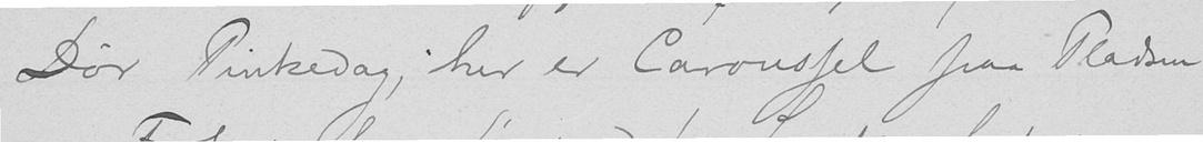Dør Pintsedag; her er Caroussel paa Pladsen
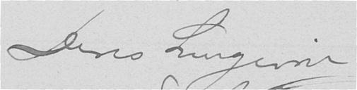Deres hengivne
